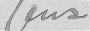Jens
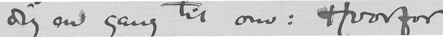dig en gang til om: Hvorfor
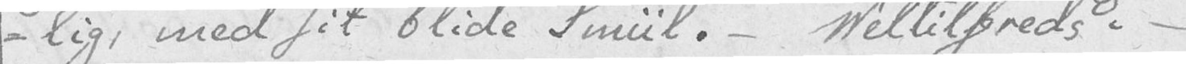-lig, med sit blide Smiil. – Veltilfreds. –
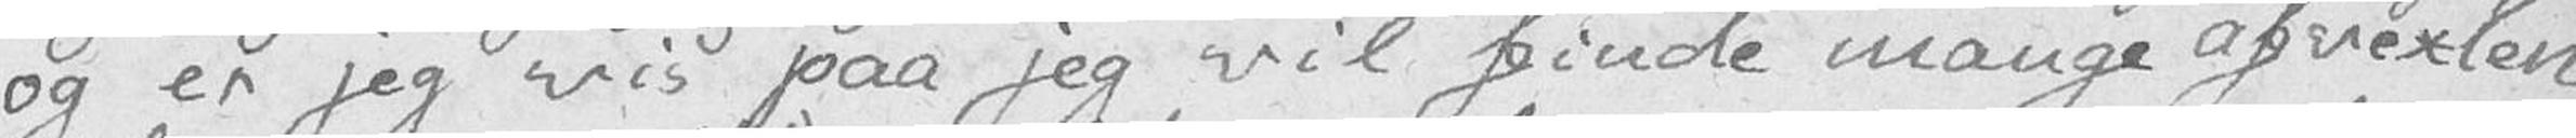og er jeg vis paa jeg vil finde mange afvexlen
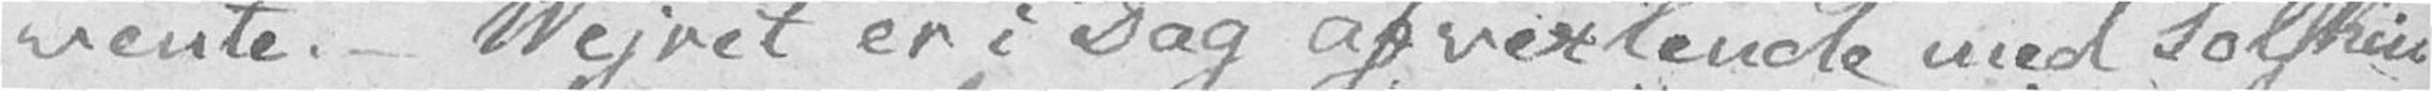vente. Vejret er i Dag afvexlende med Solskin
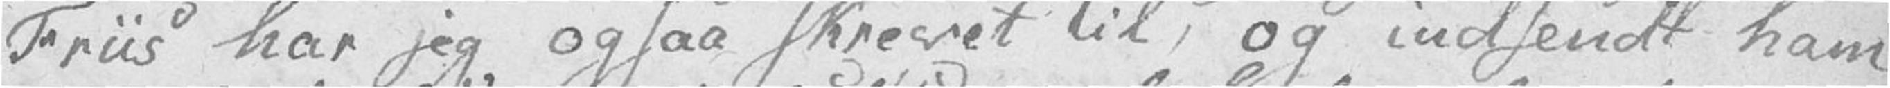Friis har jeg ogsaa skrevet til, og indsendt ham
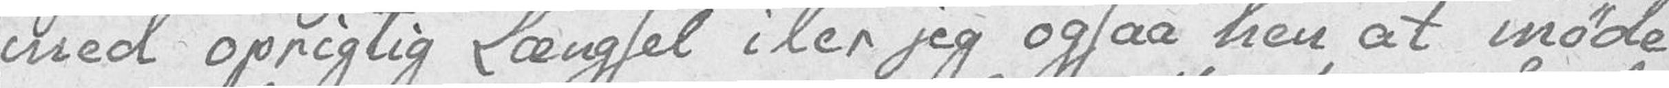med oprigtig Længsel iler jeg ogsaa hen at møde
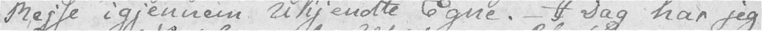Rejse igjennem Ukjendte Egne. – I Dag har jeg
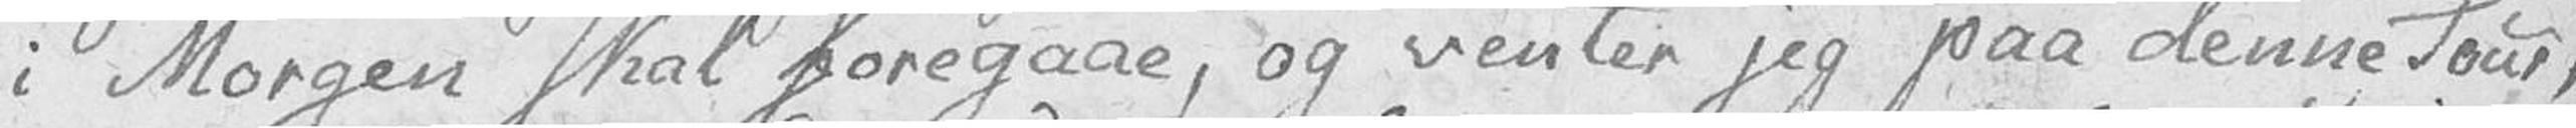i Morgen skal foregaae, og venter jeg paa denne Tour,
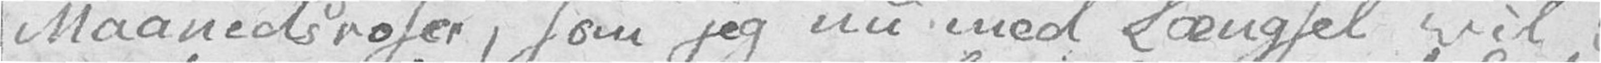Maanedsroser, som jeg nu med Længsel vil
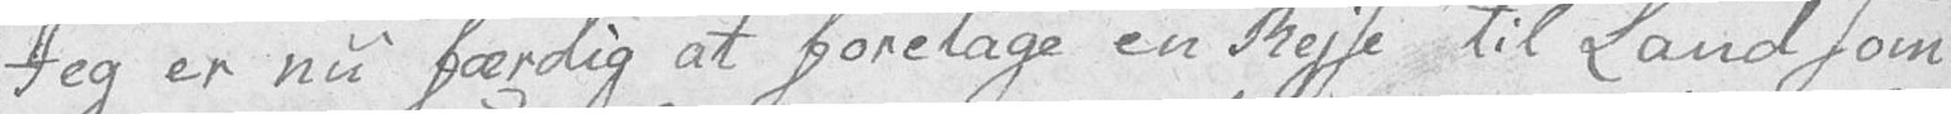Jeg er nu færdig at foretage en Rejse til Land som
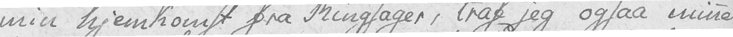min hjemkomst fra Ringsager, traf jeg ogsaa minne
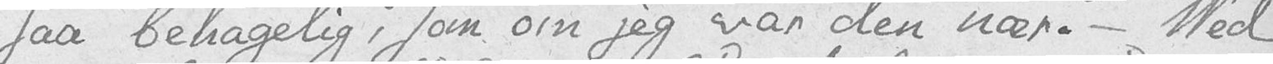saa behagelig, som om jeg var den nær. – Ved
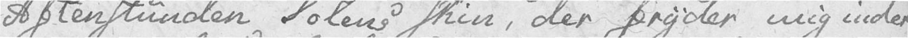Aftenstunden Solens skin, der fryder mig inder
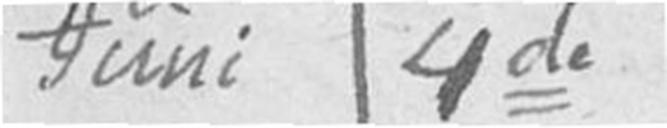Juni 4de
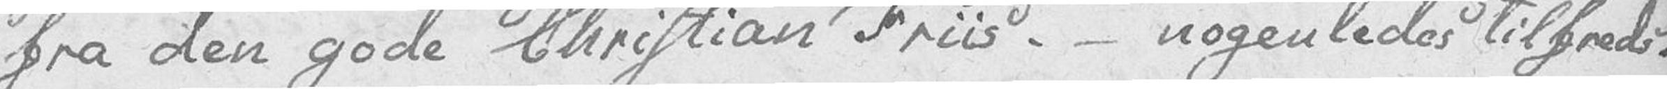fra den gode Christian Friis. – nogenledes tilfreds.
